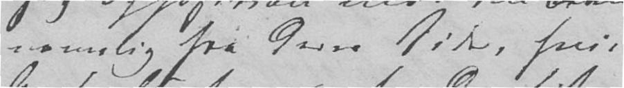nemlig fra deres Side, hvis
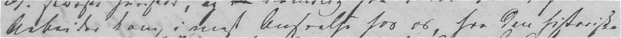Arbeider kom i meest Anseelse hos os fra den historiske
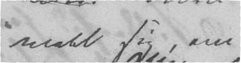maalet sig, om
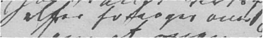eller forsagn overst
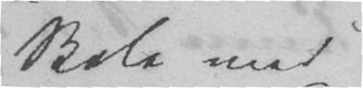Skole med
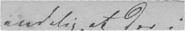endelig, at der i
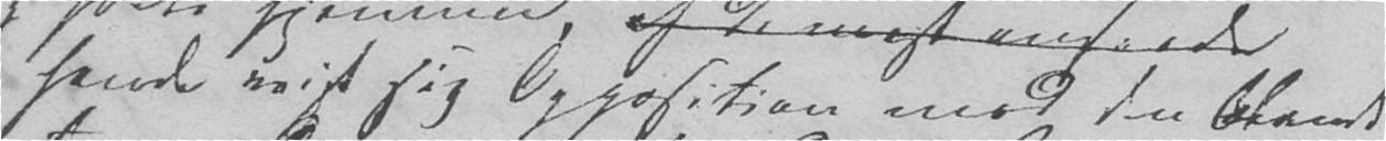havde vist sig Opposition mod den blandt
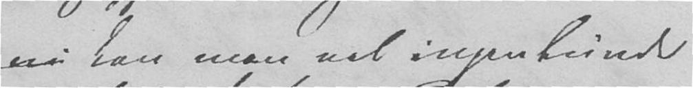kan man vel ingenlunde
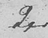Der
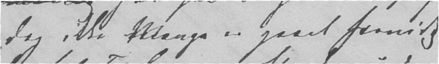dog ikke Mange er gaaet forvidt
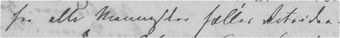for alle Mennesker fælles Retsidee.
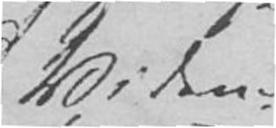Viden-
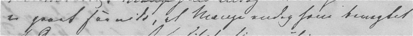er gaaet saa vidt, at Mange endog have benægtet
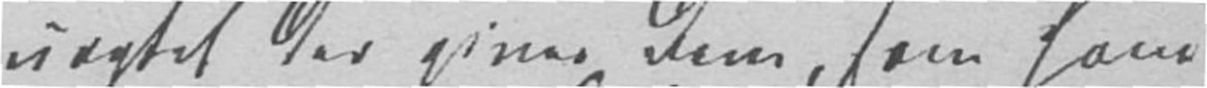uagtet der gives dem, som have
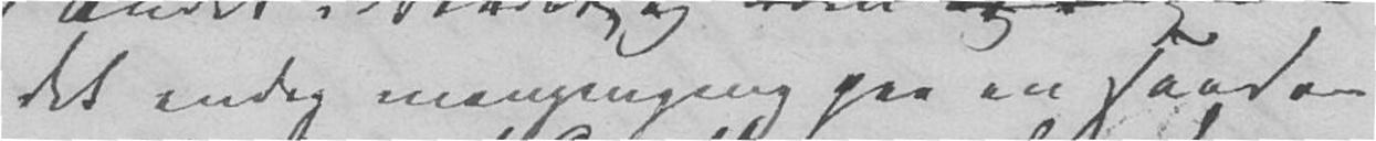det endog mangengang paa en saadan
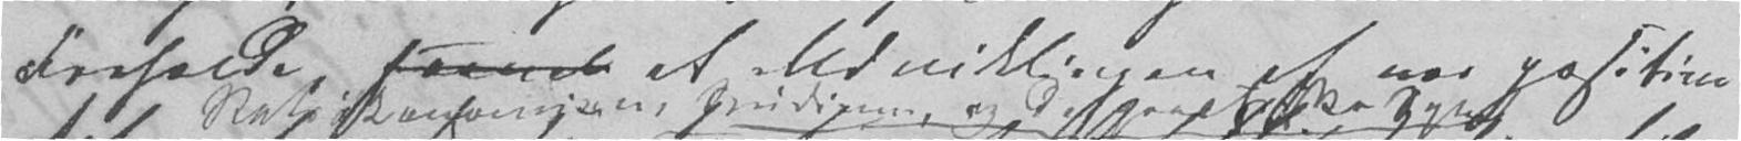Forholde, at Udviklingen af vor positive
